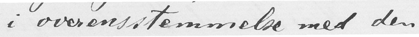i overensstemmelse med den
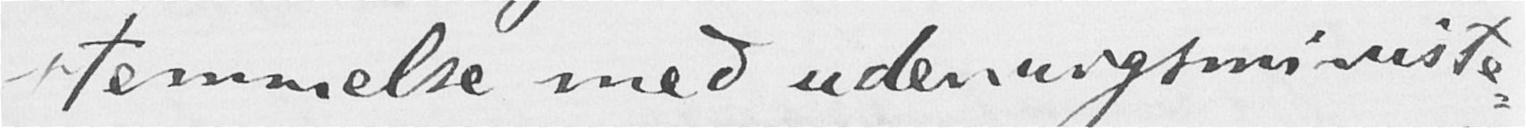stemmelse med udenrigsministe-
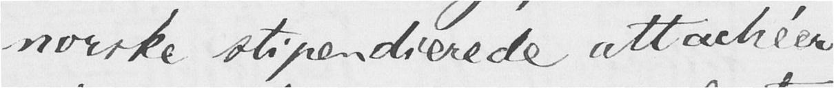norske stipendierede attachéer
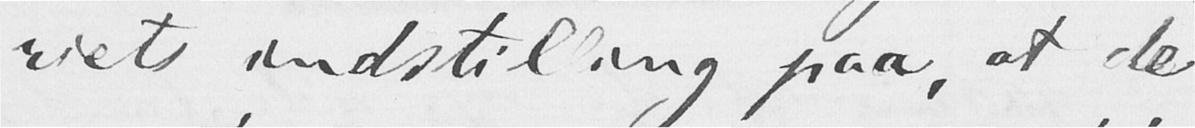riets indstiling paa, at de
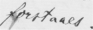forstaaes.
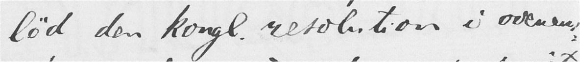lød den kongl. resolution i overens-
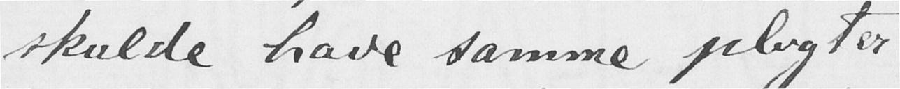skulde have samme pligter
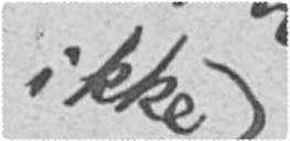ikke
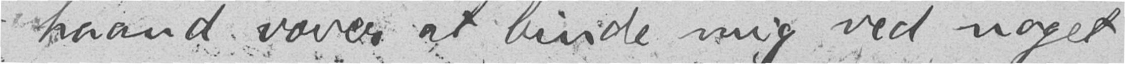haand vover at binde mig ved noget
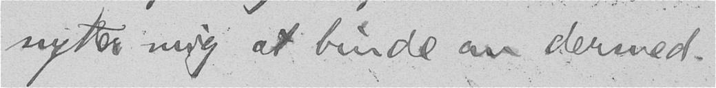nytter mig at binde an dermed.
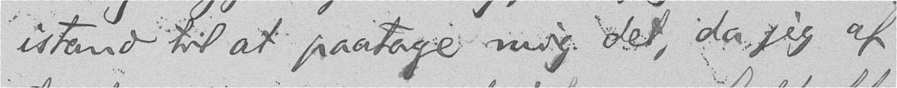istand til at paatage mig det, da jeg af
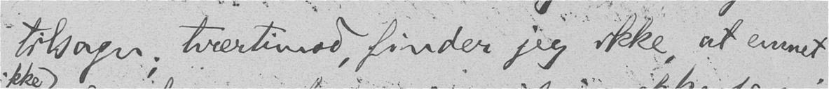tilsagn; tværtimod, finder jeg ikke, at emnet
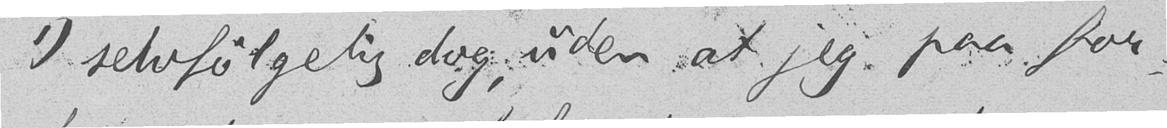1) selvfølgelig dog, uden at jeg paa for-
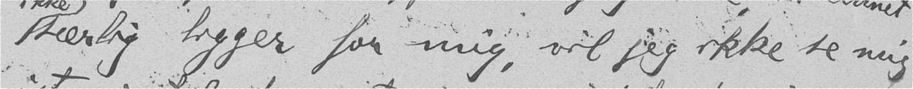særlig ligger for mig, vil jeg ikke se mig
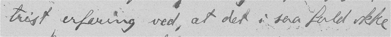trist erfaring ved, at det i saa fald ikke
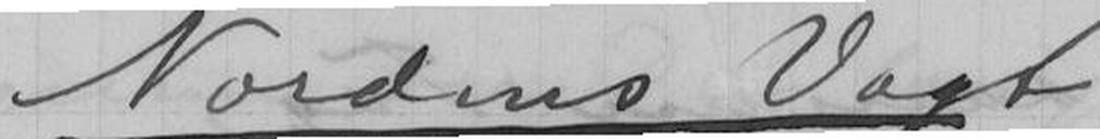Nordens Vagt
